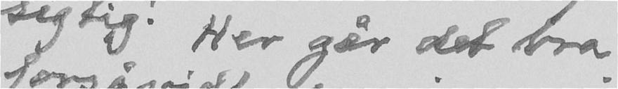sigtig! Her går det bra
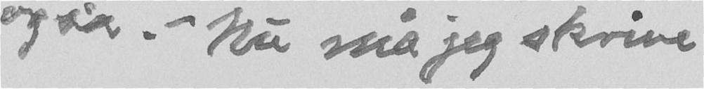også. - Nu må jeg skrive
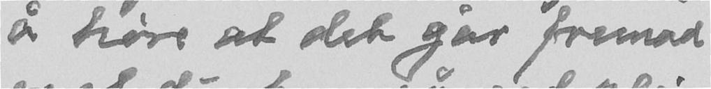å høre at det går fremad
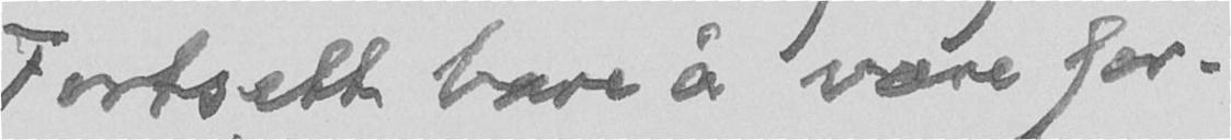Fortsett bare å være for-
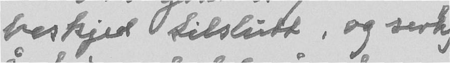beskjed tilslutt, og særlig
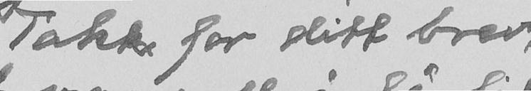Takk for ditt brev,
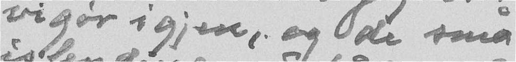vigør igjen, og de små
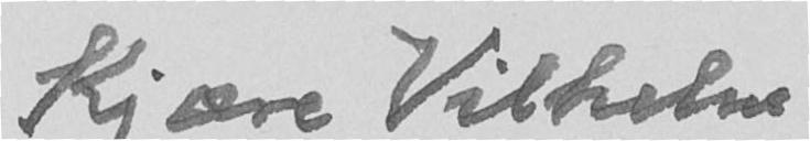Kjære Vilhelm,
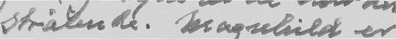strålende. Magnhild er
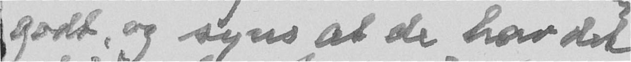godt og syns at de har det
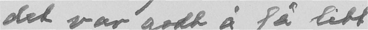det var godt å få litt
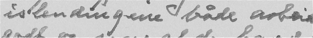islendingene både arbeider
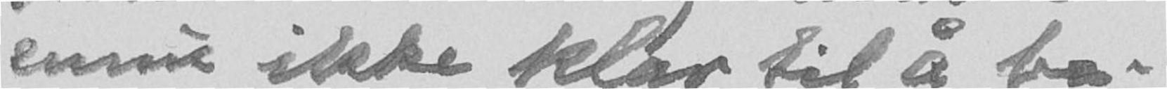ennu ikke klar til å be-
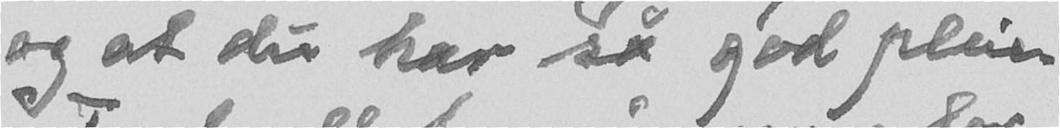og at du har så god pleie.
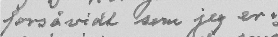forsåvidt som jeg er i
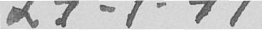29-1-47
